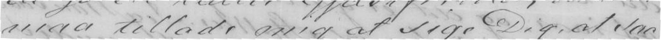maa tillade mig at sige Dig, at saa
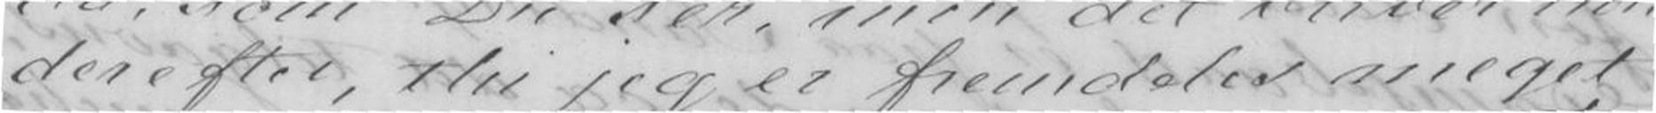derefter, thi jeg er fremdeles meget
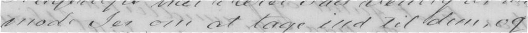mode Jer om at tage ind til dem, og
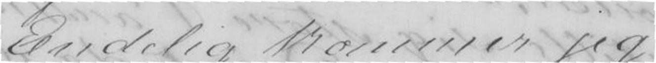Endelig kommer jeg
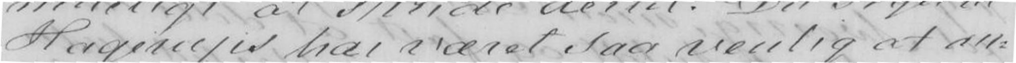Hagerups har været saa venlig at an-
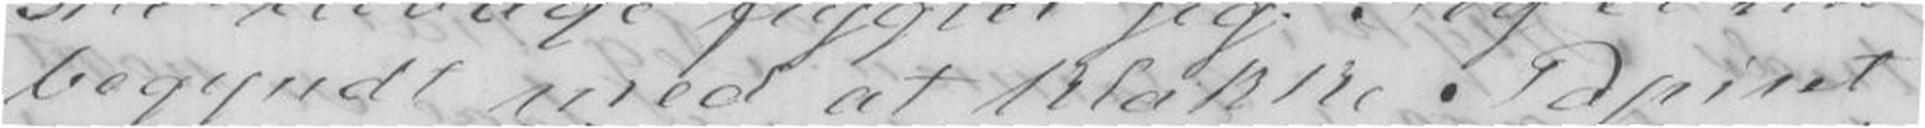begyndt med at kladde Papiret
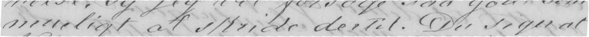muelig at skride dertil. Du siger at
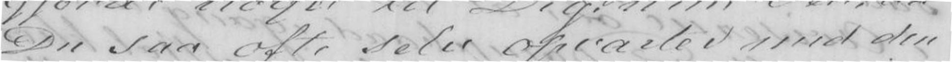Du saa ofte selv opvarter med den
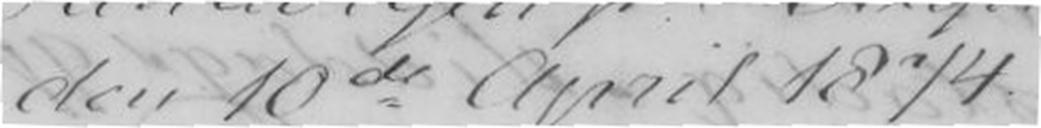den 10de April 1874.
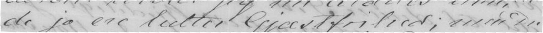de jo ere lutter Gjæstfrihed; men Du
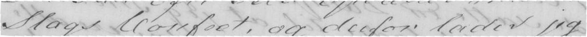Slags Confect, og derfor lader jeg
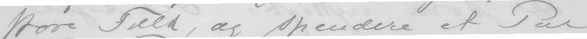store Folk, og spendere at Par
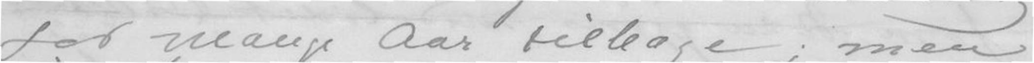for mange Aar tilbage; men
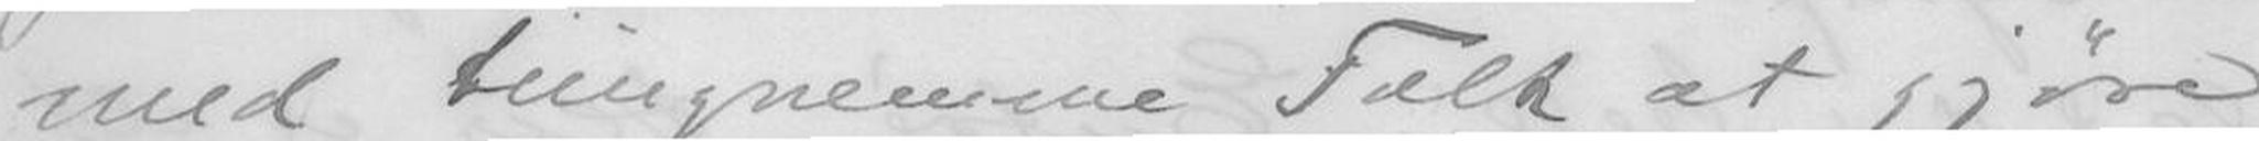med tungnemme Folk at gjøre,
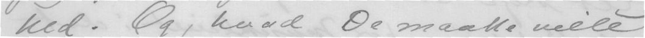hed. Og, hvad De maatte ville
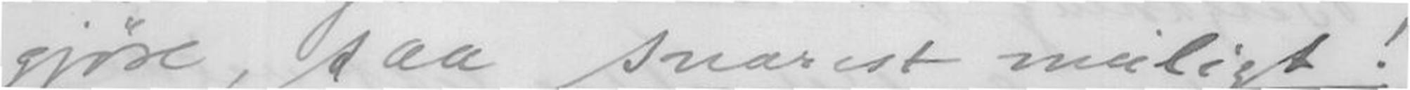gjøre, saa snarest muligt!
